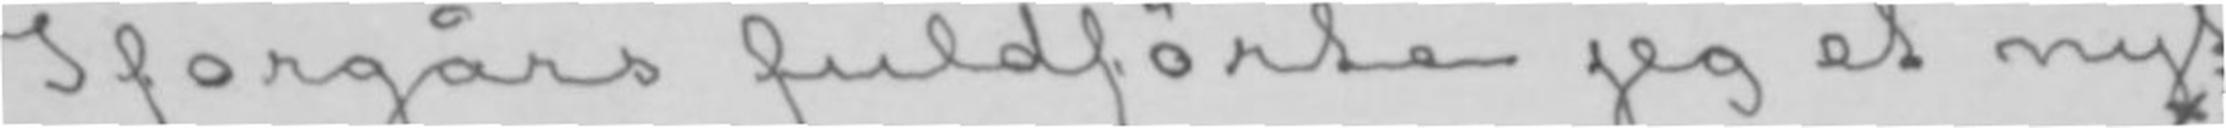Iforgårs fuldførte jeg et nyt
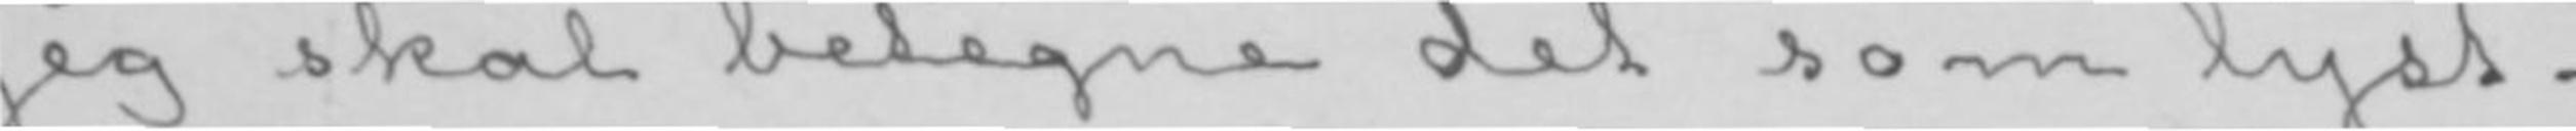jeg skal betegne det som lyst-
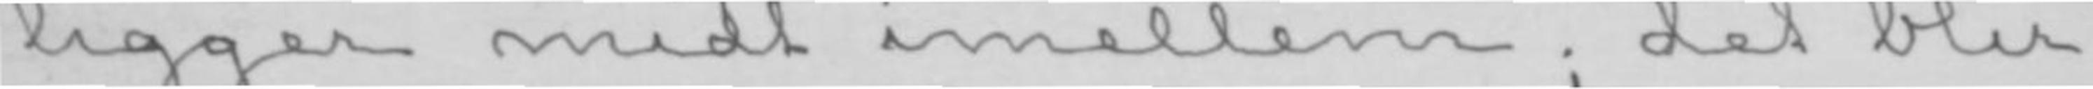ligger midt imellem; det blir
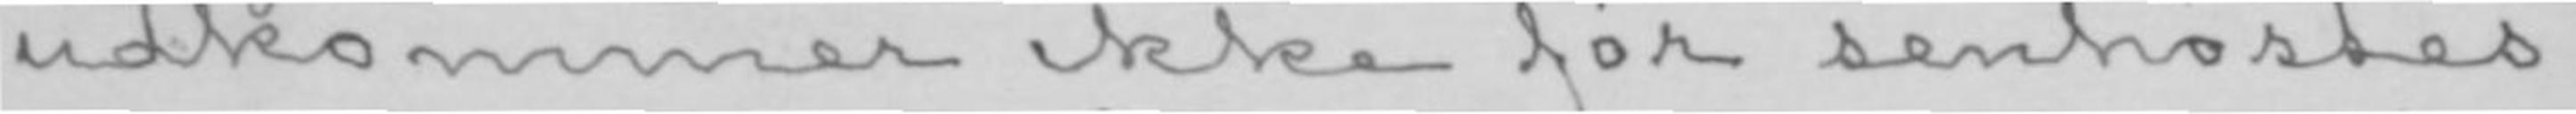udkommer ikke før senhøstes.
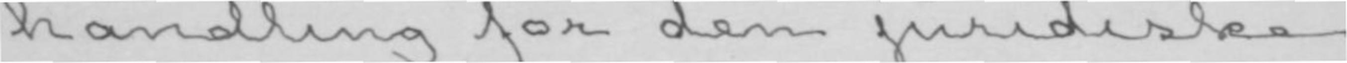handling for den juridiske
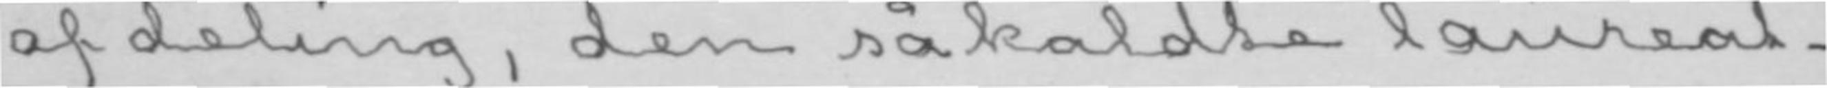afdeling, den såkaldte laureat-
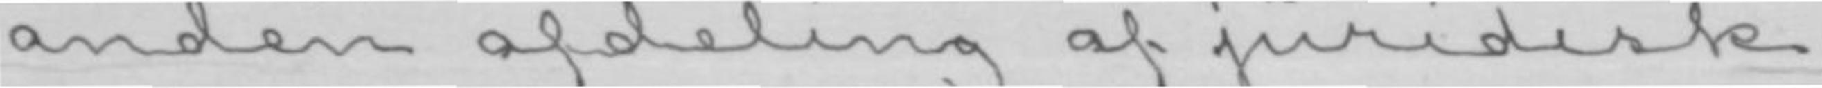anden afdeling af juridisk
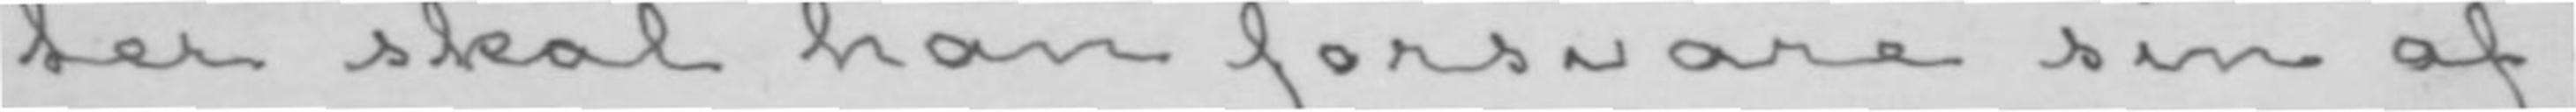ter skal han forsvare sin af-
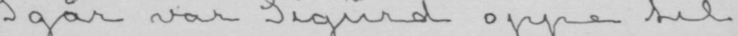Igår var Sigurd oppe til
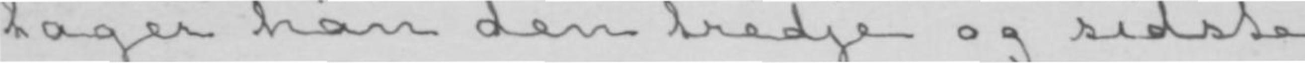tager han den tredje og sidste
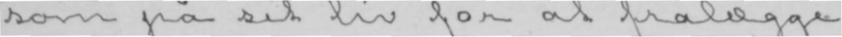som på sit liv for at fralægge
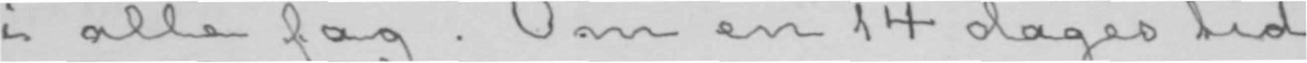i alle fag. Om en 14 dages tid
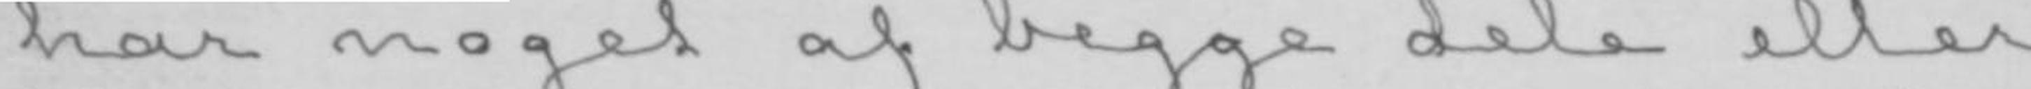har noget af begge dele eller
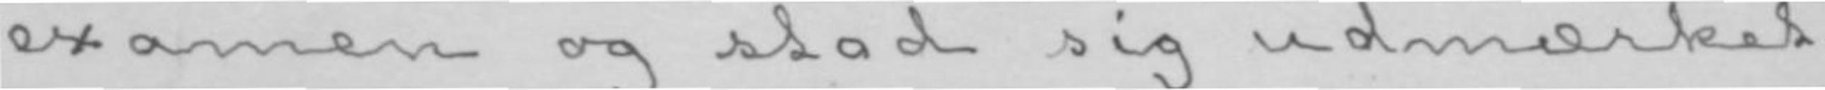examen og stod sig udmærket
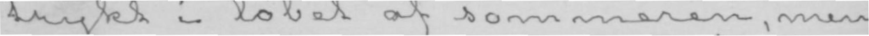trykt i løbet af sommeren, men
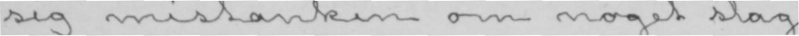sig mistanken om noget slags
